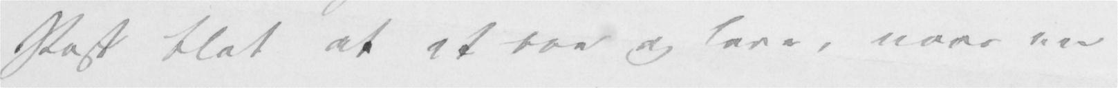Post blot at at boe og leve, naar nu
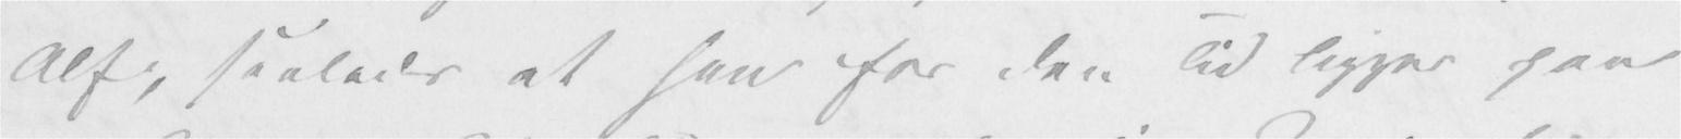Alf, saaledes at han for den Tid ligger paa
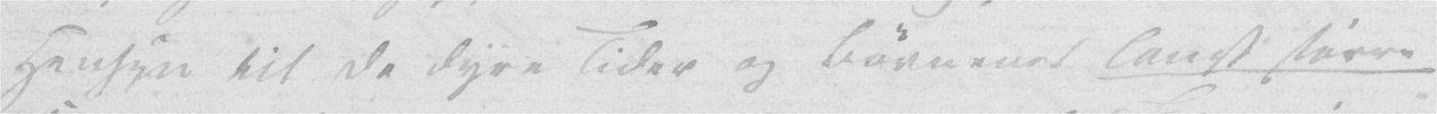Hensyn til de dyre Tider og Børnenes langt større
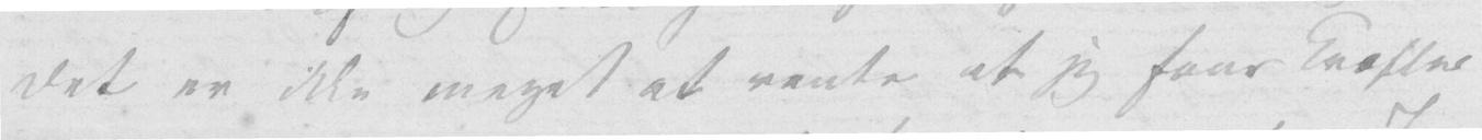det er ikke meget at vente at jeg faar Kræfter
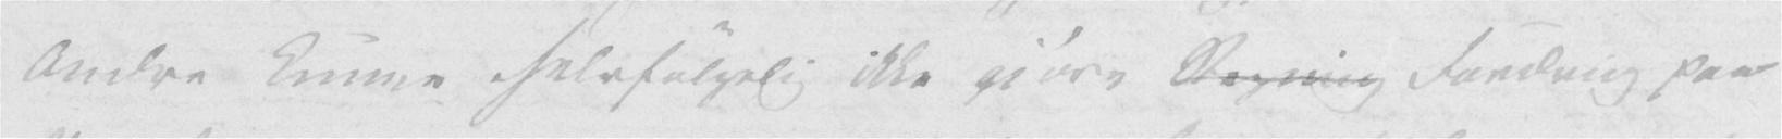Andre kunne selvfølgelig ikke gjøre Regning Fordring paa
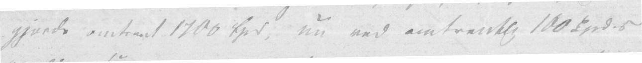udgjorde omtrent 1700 Spd, nu ved omtrentlig 100 Spd.s
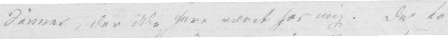Sønner, der ikke have været hos mig. De to
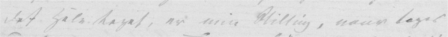det Hele taget, er min Stilling, naar tages
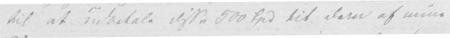til at udbetale disse 500 Spd til dem af mine
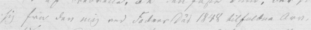sig fra den mig ved Faders Død 1848 tilfaldne Arv,
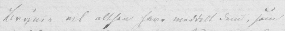Brynie vil altsaa have meddelt Dem, som
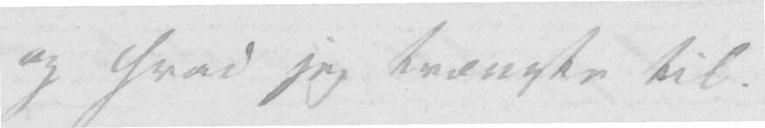og hvad jeg trængte til.
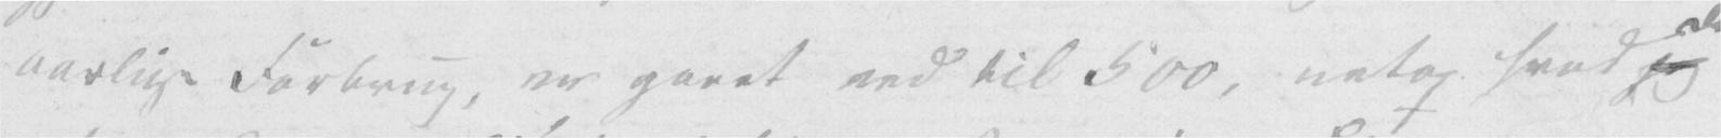aarlige Forbrug, er gaaet ned til 500, netop hvad jeg
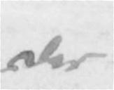der
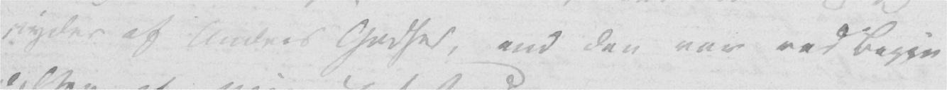nyder af Andres Godhed, end den var ved Begyndelsen
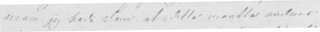maa jeg bede Dem at dette maatte ordnes
